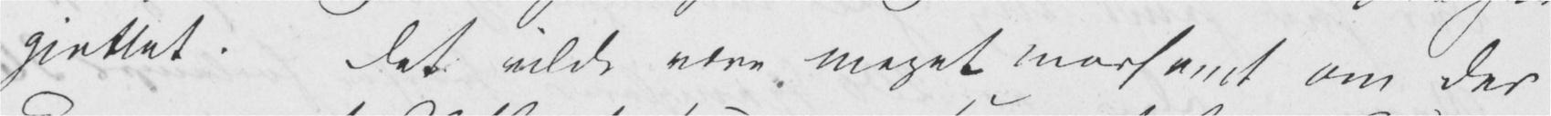gjettet. Det vilde være meget morsomt om der
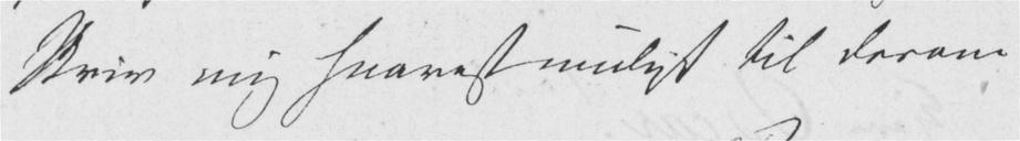Skriv mig snarest muligt til derom
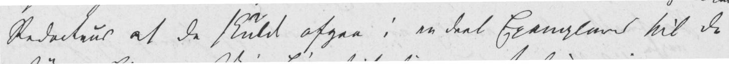Redacteur at de skulde afgaa i en deel Exemplarer til de
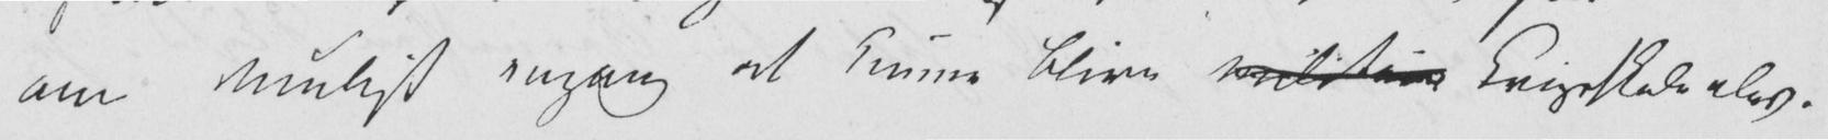om muligt engang at kunne blive militair Krigsskoleelev.
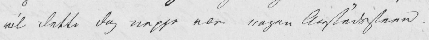vil dette dog neppe være nogen Anstødssteen.
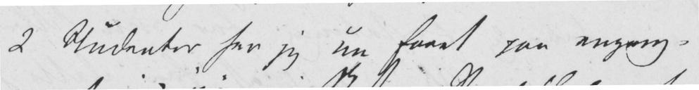2 Studenter har jeg nu faaet paa engang,
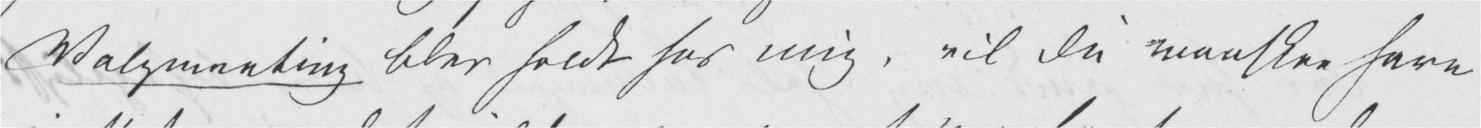Valgmanting blev holdt hos mig, vil Du maaske have
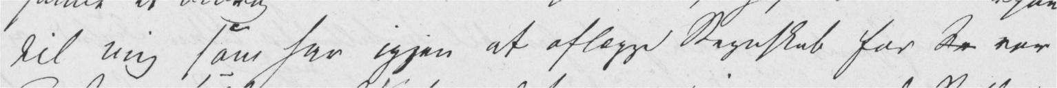til mig som har igjen at aflægge Regnskab for Se vor
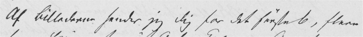Af Billederne sender jeg Dig for det første 6, flere
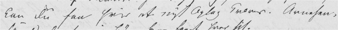kan Du faa hvis et nyt Oplag kræves. Arnesen,
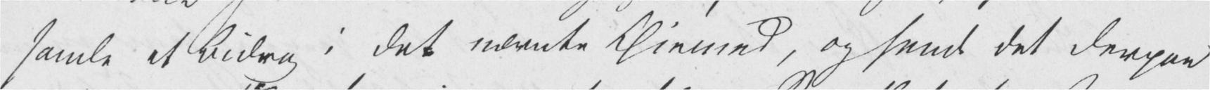samle et Bidrag i det nævnte Øiemed, og sende det derpaa
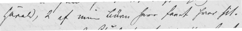Harald, 2 af mine Børn have faaet hver sit.
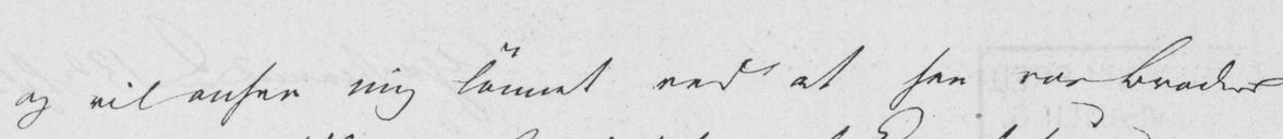og vil ansee mig lønnet ved at see vor Broders
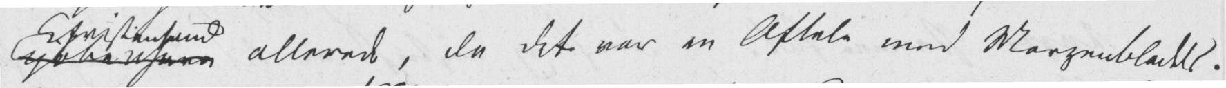Kjøbenhavn allerede, da det var en Aftale med Morgenbladets
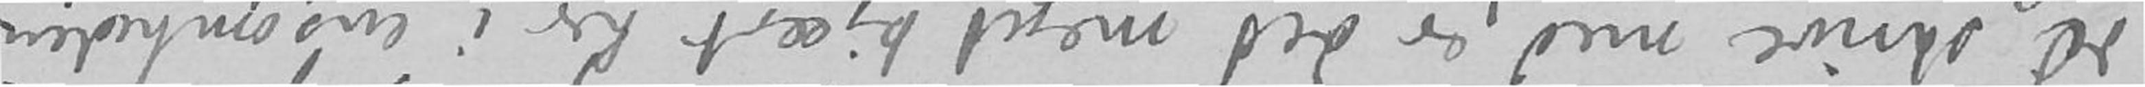de skrive med, er det meget kjært her i ensomheden.
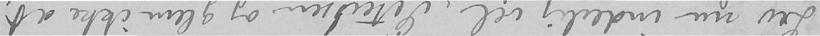Lev nu inderlig vel, Peterssen og glem ikke at
